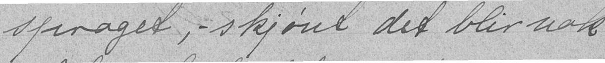sproget, - skjønt det blir nok
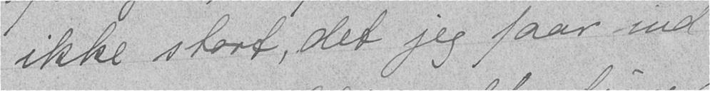ikke stort, det jeg faar ind
